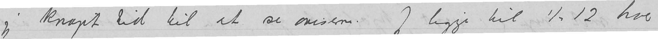jeg knapt tid til at se aviserne.  Jeg ligger til ½ 12 hver
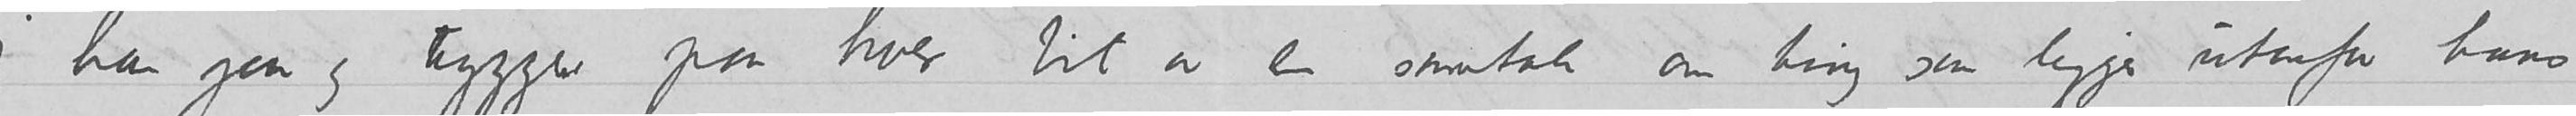jeg han gaar og tygger paa hver bit av en samtale om ting som ligger utenfor hans
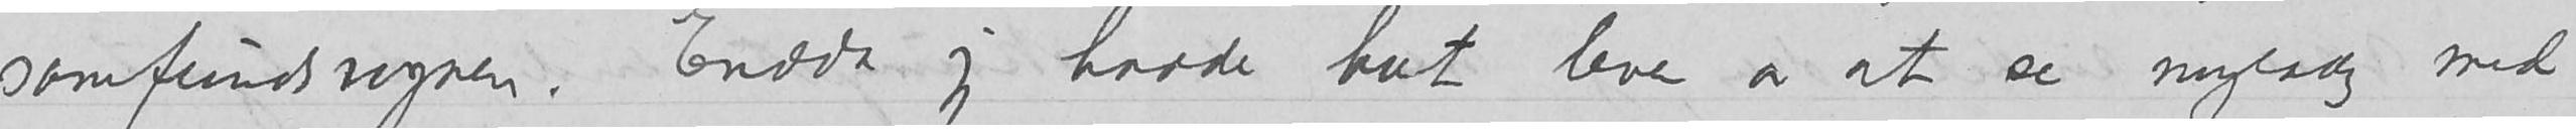samfundsvognen. Endda jeg hadde hat leven av at se mylady med
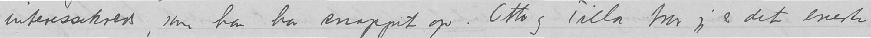interessekreds, som han har snappet op.. Otto og Tilla tror jeg er det eneste
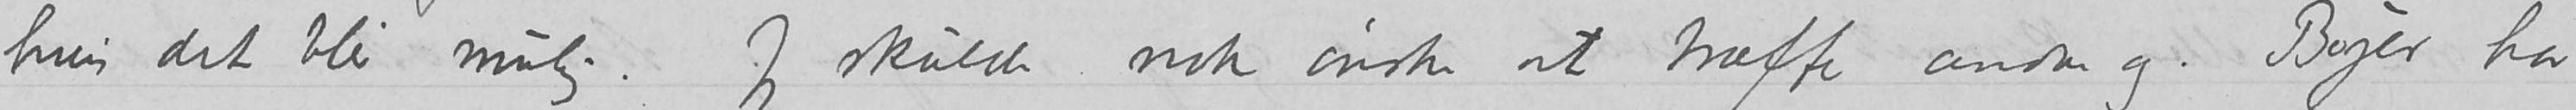hvis det blir mulig. Jeg skulde nok ønske at træffe andre og.  Bojer har
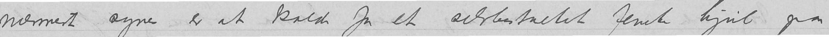nærmest synes er at kalde for et selvbestaltet femte hjul paa
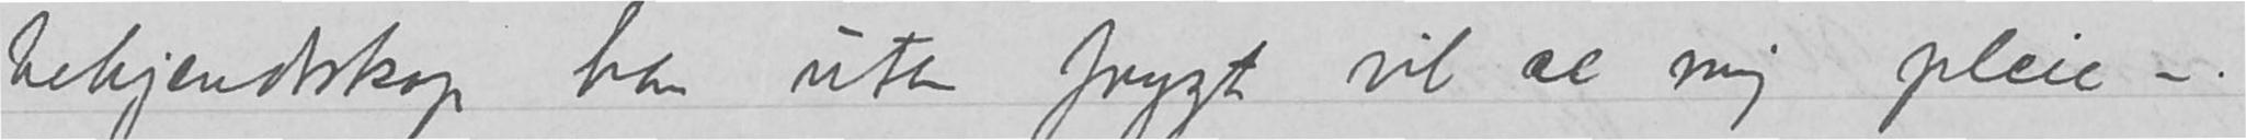bekjendskap han uten han uten frygt vil se mig pleie –
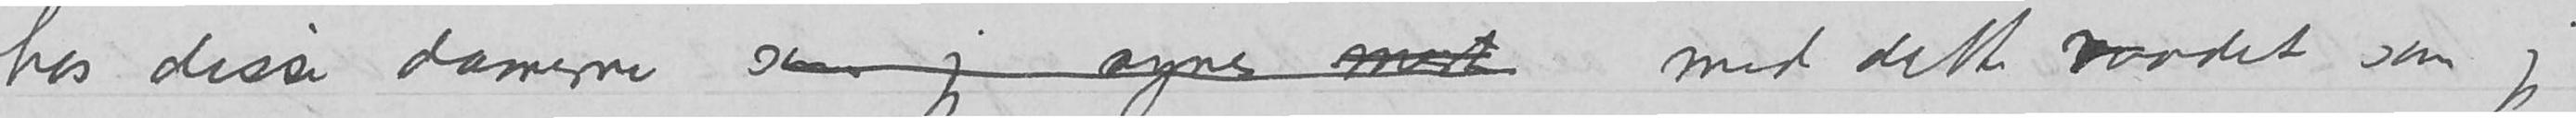hos disse damerne som jeg synes mest med dette raadet som jeg
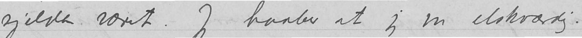sjelden været. Jeg haaber at jeg var elskværdig.
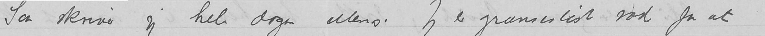Sa skriver skriver jeg hele dagen ellers.  Jeg er grænseløst ræd for at
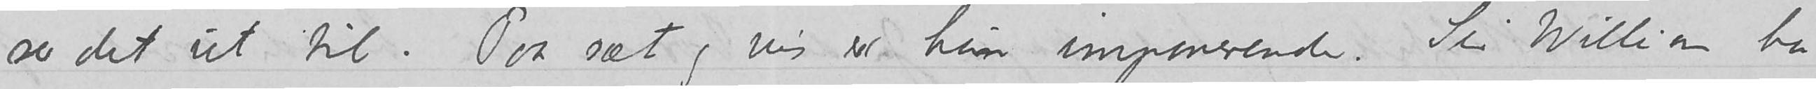ser det ut til. Paa sæt og vis er hun imponerende. Sir William har
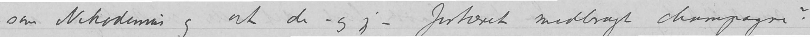som Nikodemus og at de – og jeg – fortæret medbragt champagne?
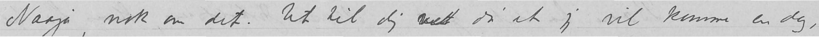Naaja, nok om det. Ut til dig vet du at jeg vil komme en dag,
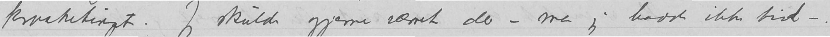kraaketinget.  Jeg skulde gjerne været der – men jeg hadde ikke tid –
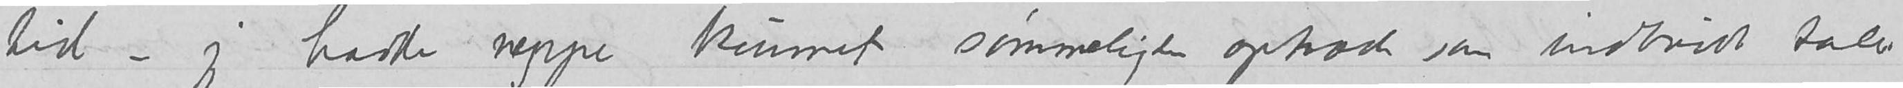tid – jeg hadde neppe kunnet sømmeligeren optræde som indbudt taler
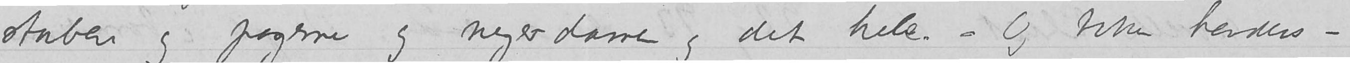staben og pagerne og negerdamer og det hele. – Og boken talen-
